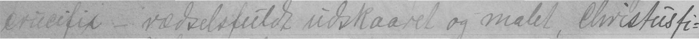crusifix - rædselsfuldt udskaaret og malet, Christusfi-
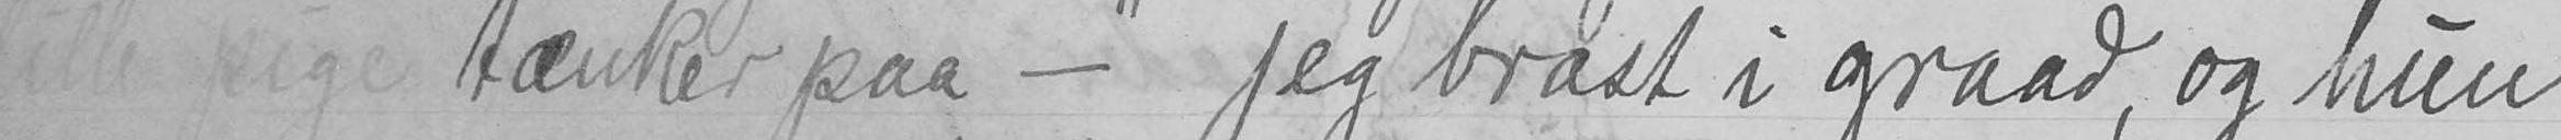lille pige tænker paa -" jeg brast i graad, og hun
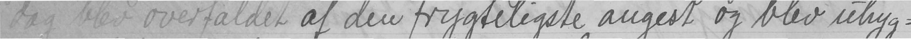dag blev overfaldet af den frygteligste angest og blev uhyg-
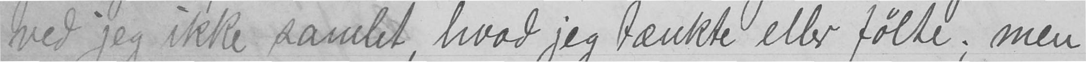ved jeg ikke samlet, hvad jeg tænkte eller følte; men
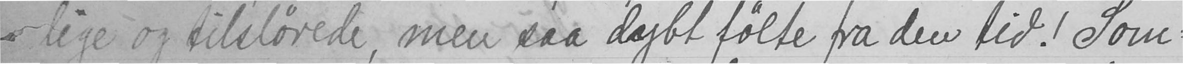lige og tilslørede, men saa dybt følte fra den tid! Som=
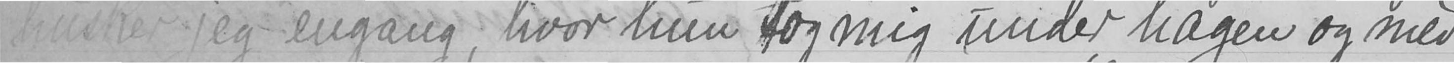husker jeg engang, hvor hun tog mig under hagen og med
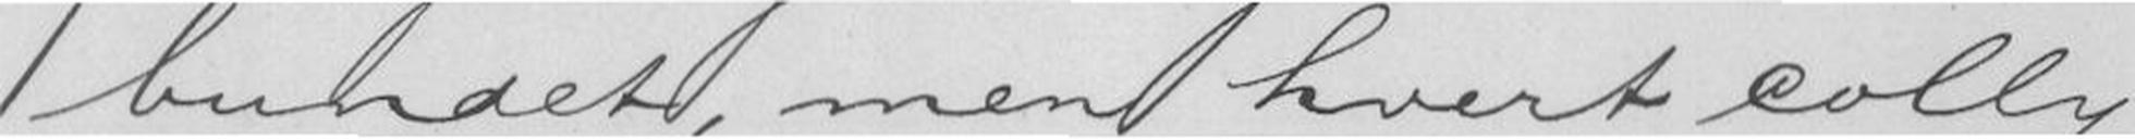bundet, men hvert colly
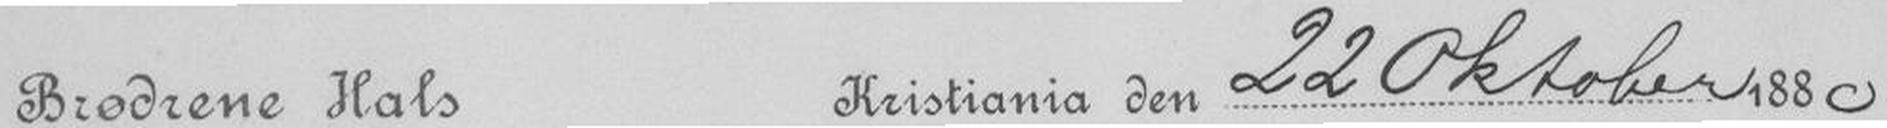Brødrene Hals Kristiania den 22 Oktober 1880
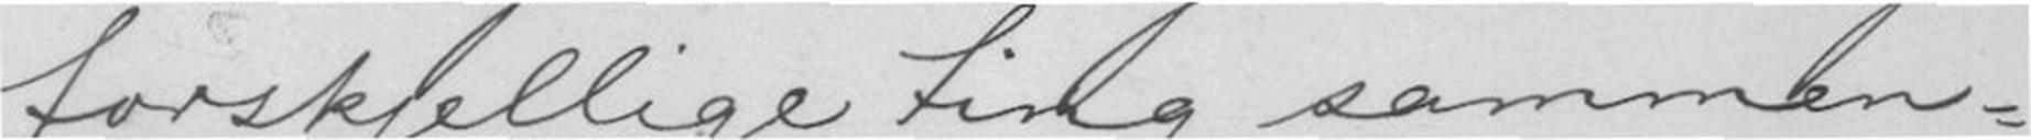forskjellige ting sammen-
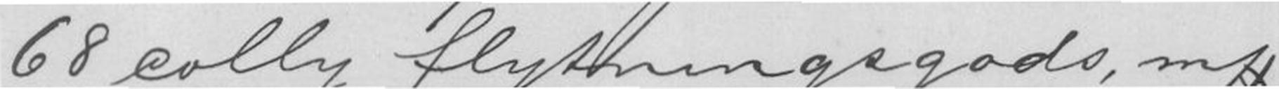68 colly flytningsgods mff
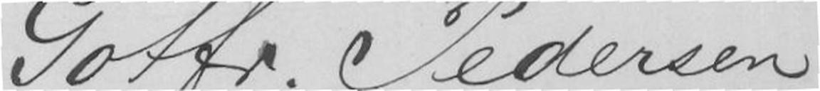Gotfr. Pedersen
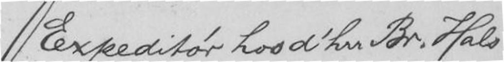Expeditør hos d'hrr Br. Hals
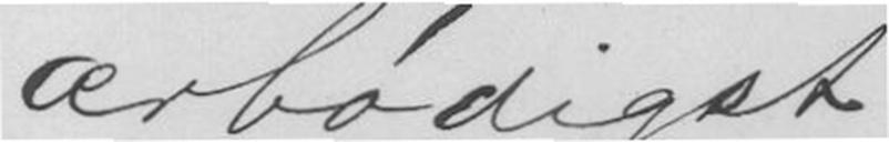ærbødigst
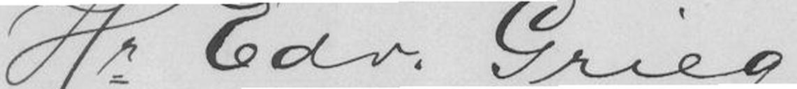Hr Edv. Grieg
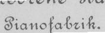Pianofabrik.
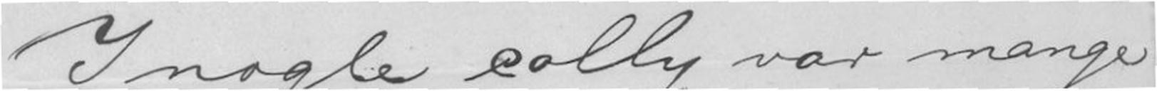I nogle colly var mange
